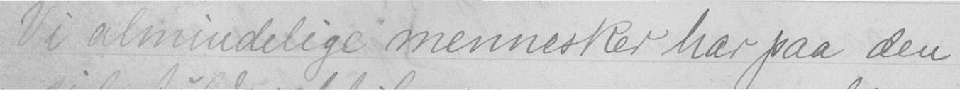Vi almindelige mennesker har paa den
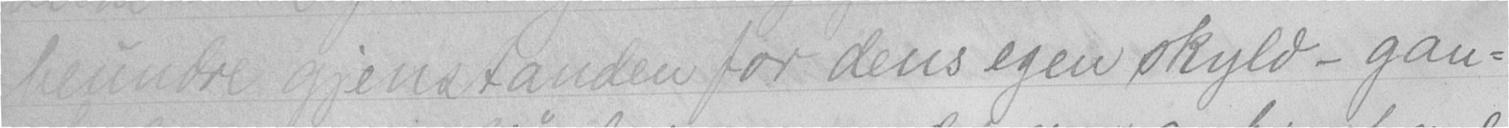beundre gjenstanden for dens egen skyld - gan-
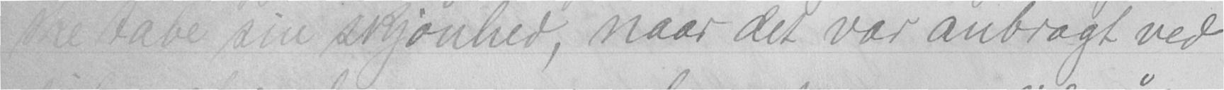ske tabe sin skjønhed, naar det var anbragt ved
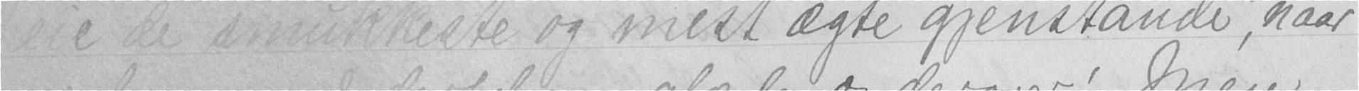eie de smukkeste og mest ægte gjenstande, naar
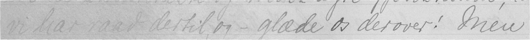vi har gaad dertil, og - glæde os derover! Men
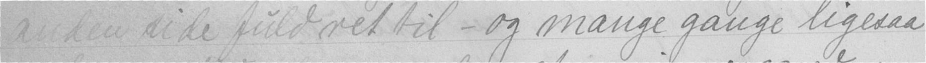anden side fuld ret til - og mange gange ligesaa
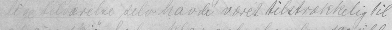lige tilværelse selv havde været tilstrækkelig til
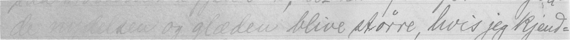de nu delsen og glæden blive større, hvis jeg kjend-
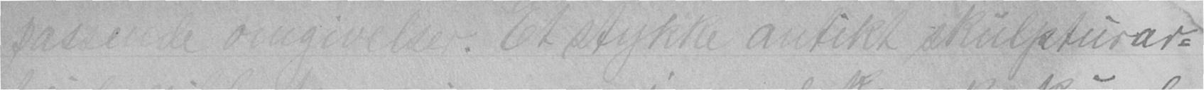passende omgivelser. Et stykke antikt skulpturar-
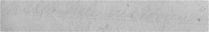nem hele historien."
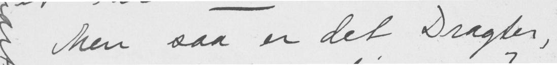Men saa er det Dragter,
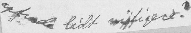optrede lidt vigtigere?
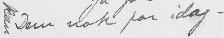Dem nok for idag -
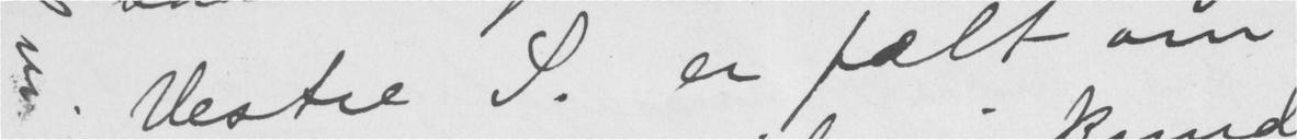Vestre S. er fælt om
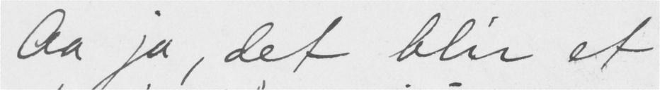Aa ja, det blir et
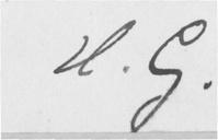H. G.
